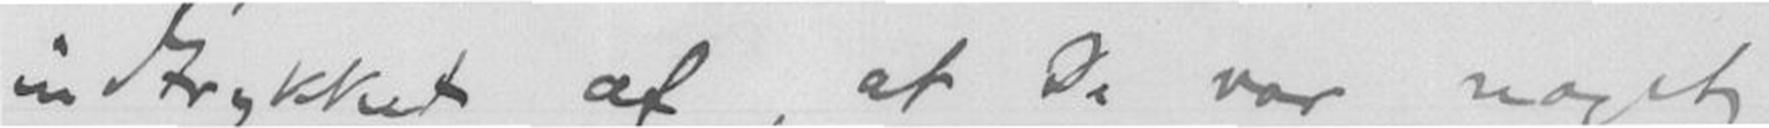indtrykket af, at Da var noget
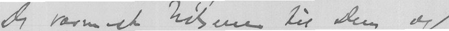De varmeste hilsener til Dem og
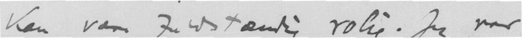kan være fuldstændig rolig. Jeg var
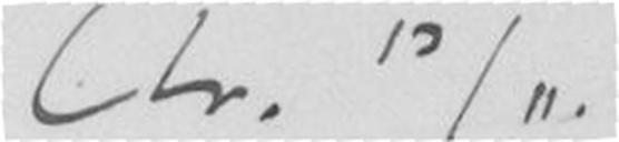Chr. 13/11.
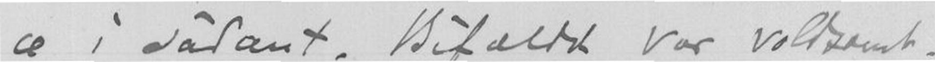ce i sådant. Bifaldet var voldsomt.
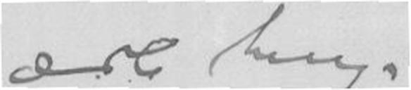ærb. heng.
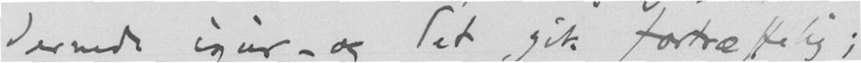dernede igår - og det gik fortræffelig;
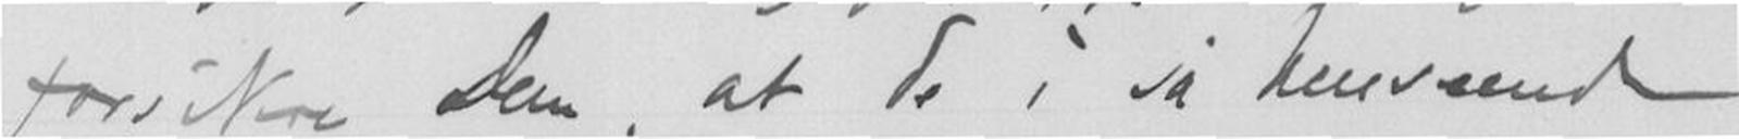forsikre Dem. at De i så hensende
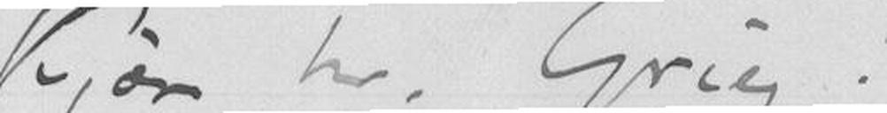Kjære hr. Grieg!
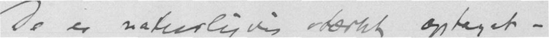De er naturligvis stærkt optaget -
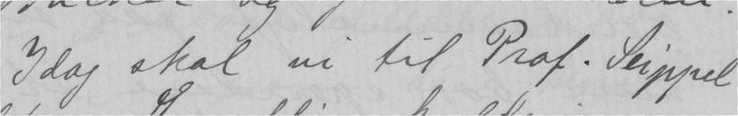Idag skal vi til Prof. Seippel
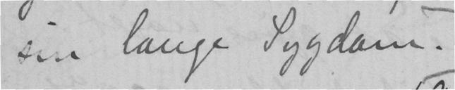sin lange sygdom.
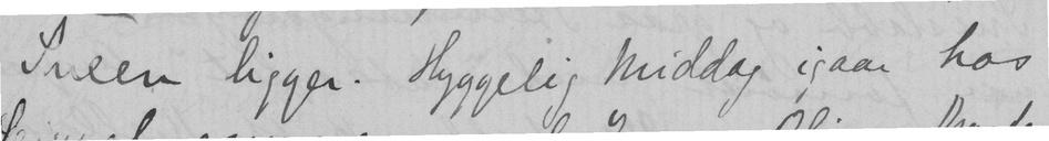Sneen ligger. Hyggelig Middag igaar hos
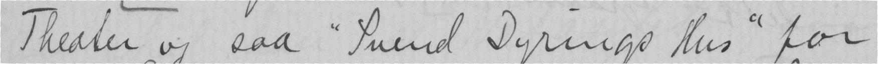Theater og saa "Svend Dyrings Hus" for
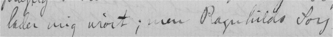lader mig urørt; men Ragnhilds Sorg
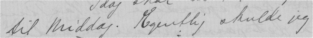til middag. Egentlig skulde jeg
